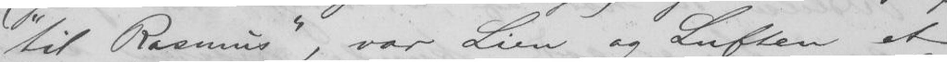"til Rasmus", var Lien og Luften et
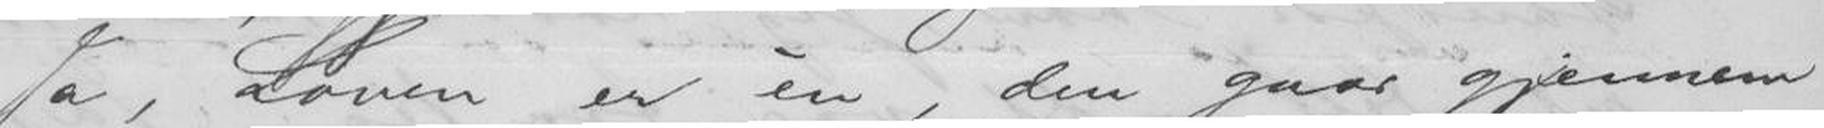Ja, Loven er én, den gaar gjennem
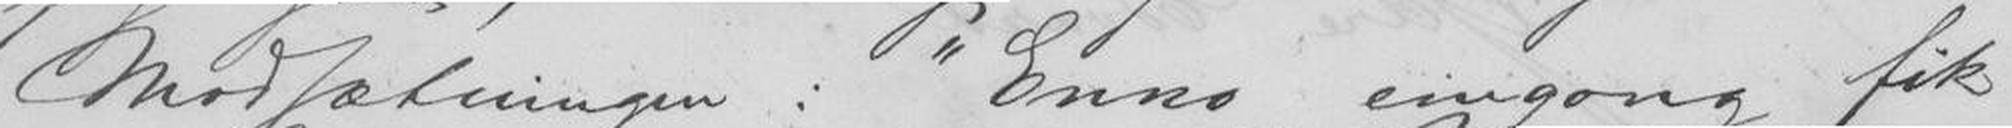Modsætningen: "Enno eingong fik
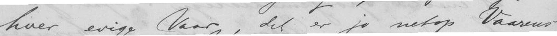hver evige Vaar, det er jo netop Vaarens
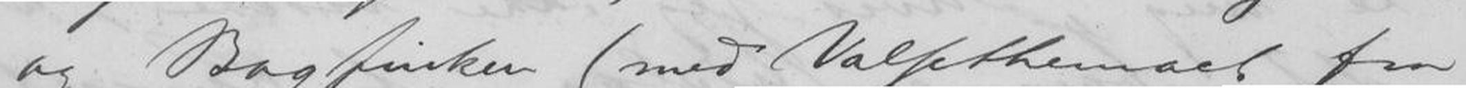og Bogfinken (med Valsethemaet fra
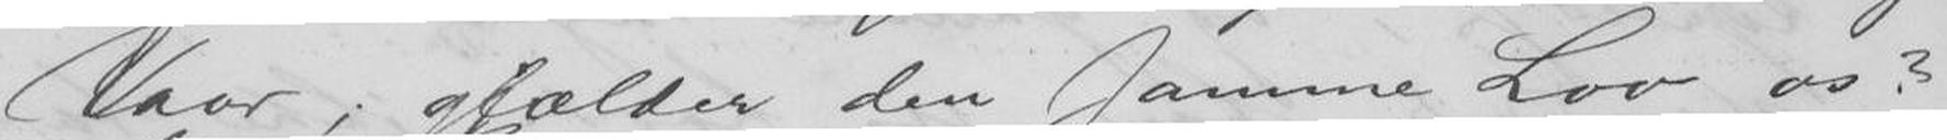Vaar; gjælder den samme Lov os?
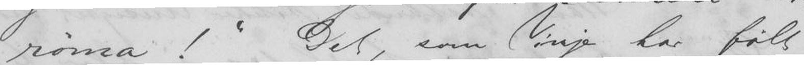røma!" Det, som Vinje har følt
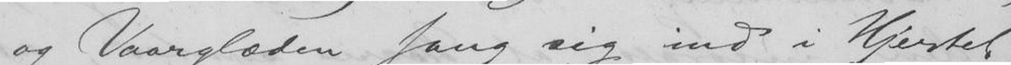og Vaarglæden sang sig ind i Hjertet
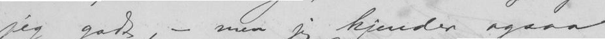jeg godt, - men jeg kjender ogsaa
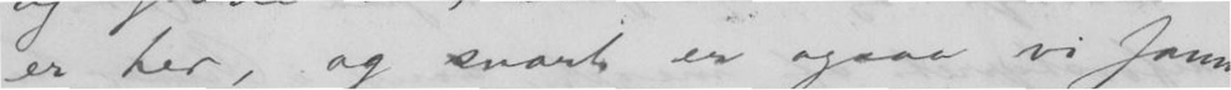er her, og snart er ogsaa vi sammen,
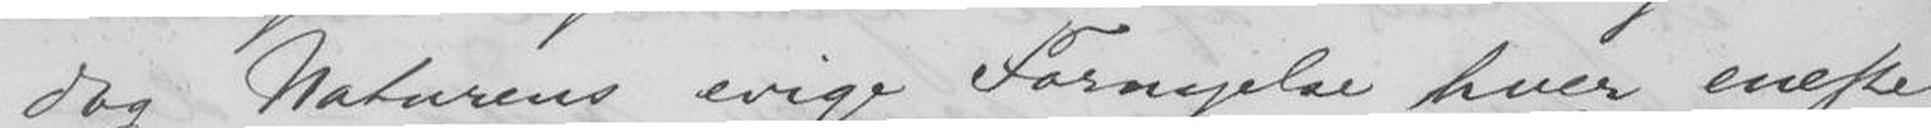dog Naturens evige Fornyelse hver eneste
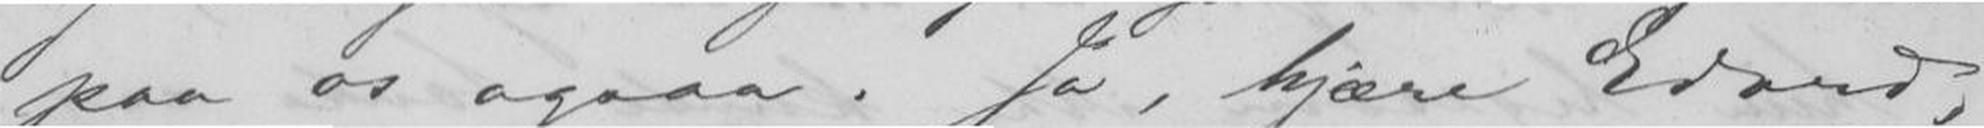paa os ogsaa. Ja, kjære Edvard,
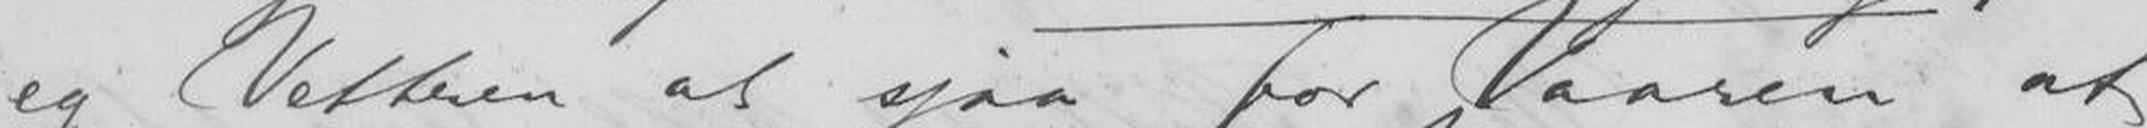eg Vettren at sjaa for Vaaren at
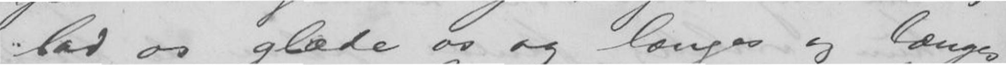lad os glæde os og længes og længes
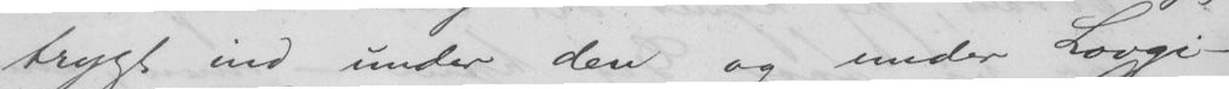trygt ind under den og under Lovgi-
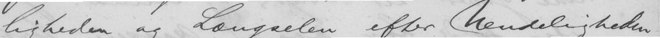ligheden og Lænselen efter Uendeligheden
